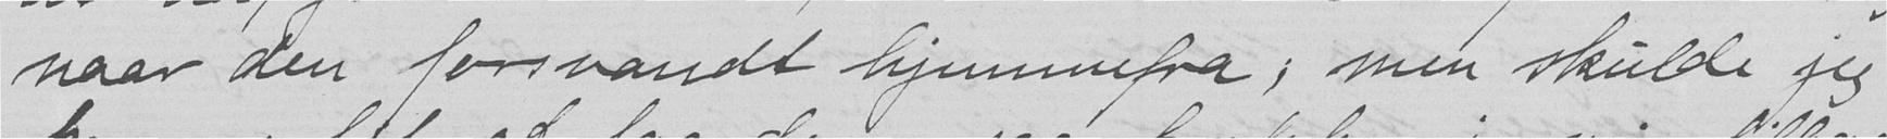naar den forsvandt hjemmefra; men skulde jeg
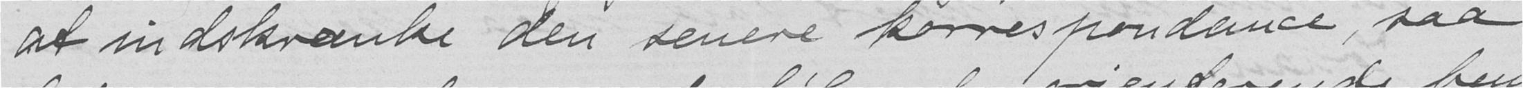at indskrænke den senere korrespondance, saa
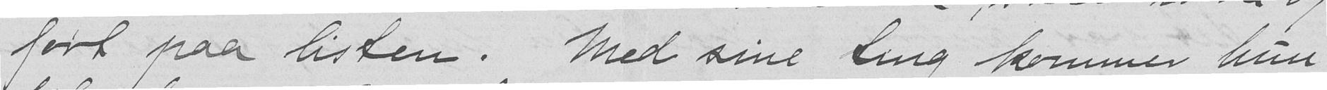ført paa listen. Med sine ting kommer hun
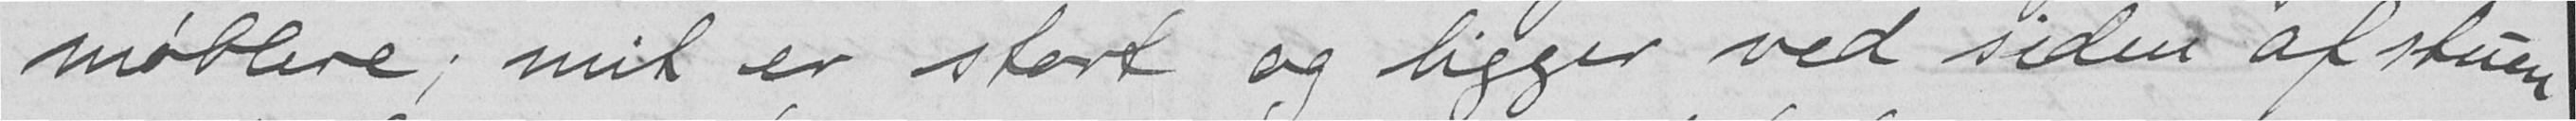møblere; mit er stort og ligger ved siden af stuen
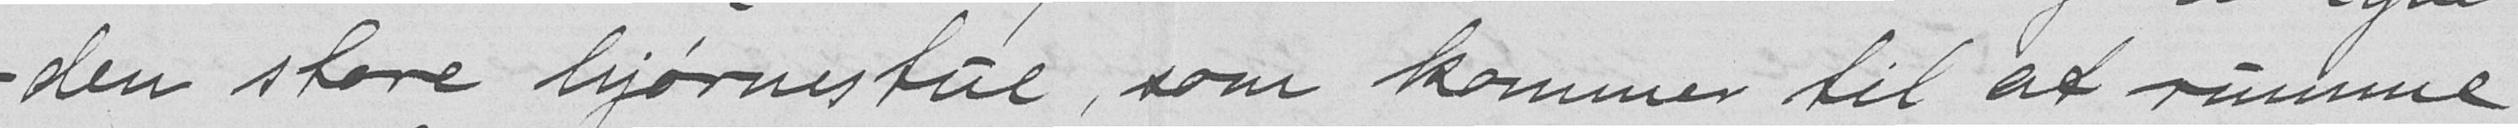- den store hjørnestue, som kommer til at rumme
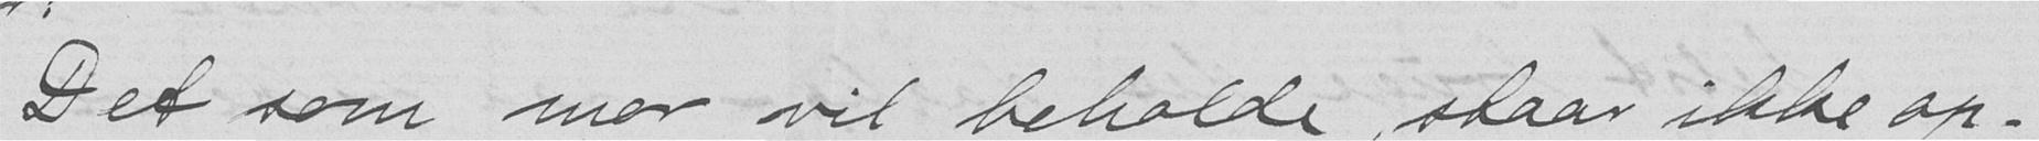Det som mor vil beholde, staar ikke op-
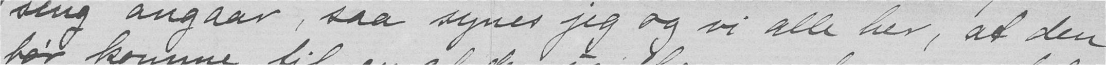seng angaar, saa synes jeg og vi alle her, at den
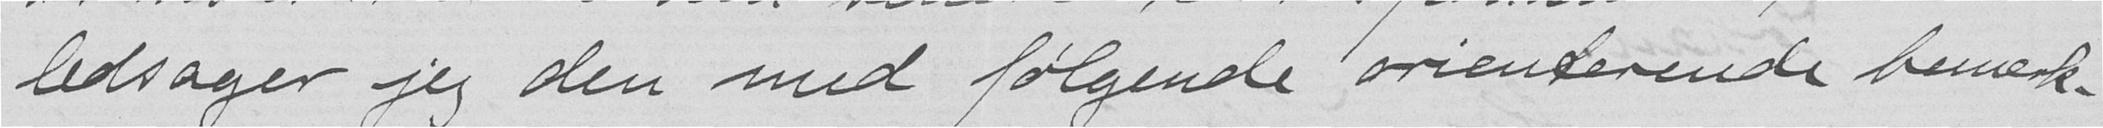ledsager jeg den med følgende orienterende bemerk-
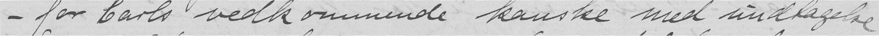- for Carls vedkommende kanske med undtagelse
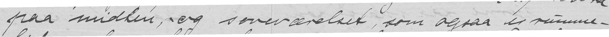paa midten, - og soveværelset, som ogsaa er rumme-
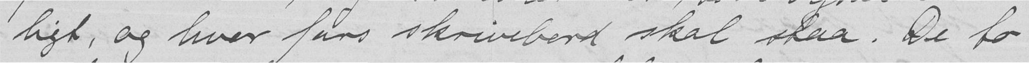ligt, og hvor fars skrivebord skal staa. De to
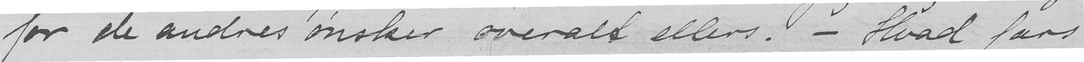for de andres ønsker overalt ellers. - Hvad fars
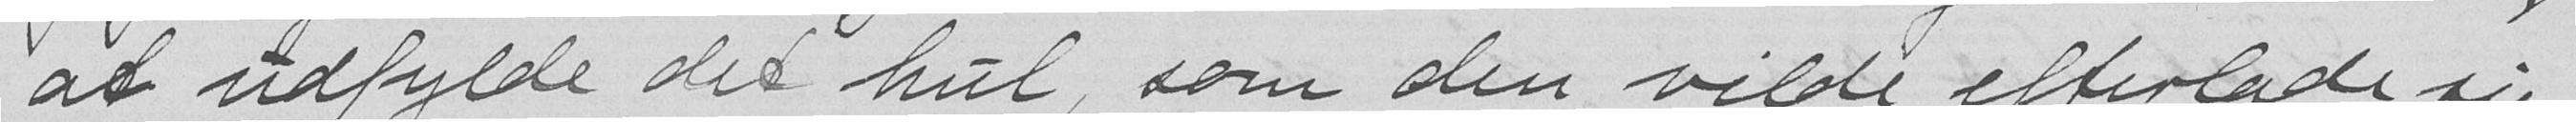at udfylde det hul, som den vilde efterlade sig
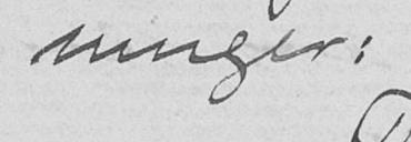ninger:
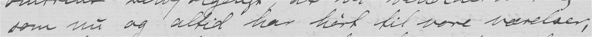som nu og altid har hørt til vore værelser,
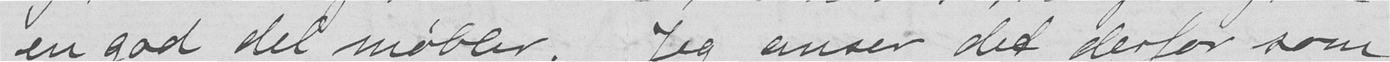en god del møbler. Jeg anser det derfor som
